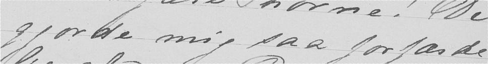gjorde mig saa for færde-
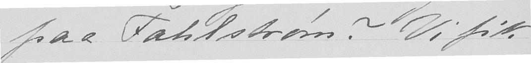paa Fahlstrøm? Vi fik
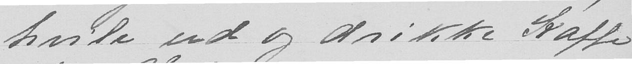hvile ud og drikke Kaffe
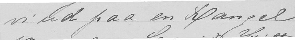vi ud paa en Rangel
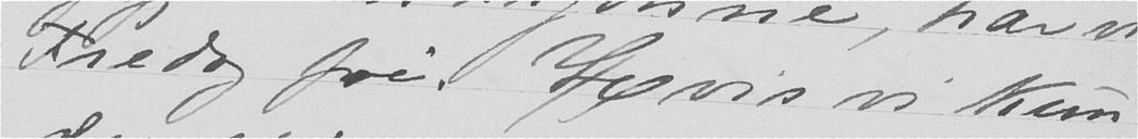Fredag fri. Hvis vi kun-
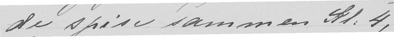de spise sammen Kl. 4,
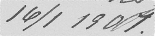16/1 1907
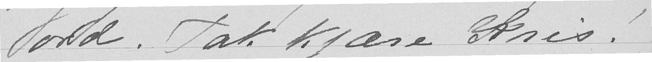ord. Tak Kjære Kris!
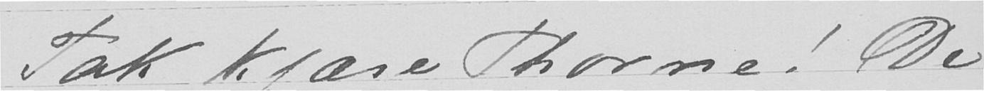Tak Kjære Thorne! De
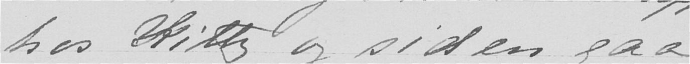hos Kitty og siden gaa
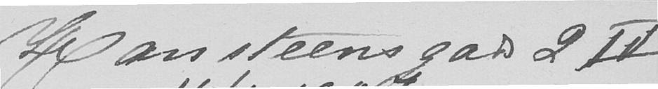Hansteens gade 2 IV
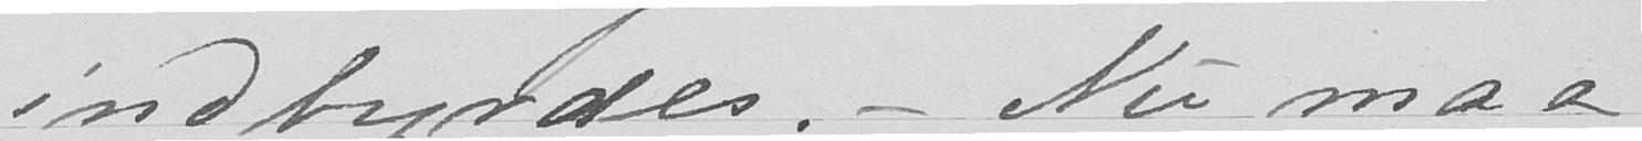indbyrdes. - Nu maa
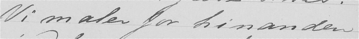Vi maler for hinanden
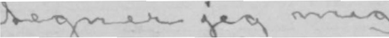tegner jeg mig
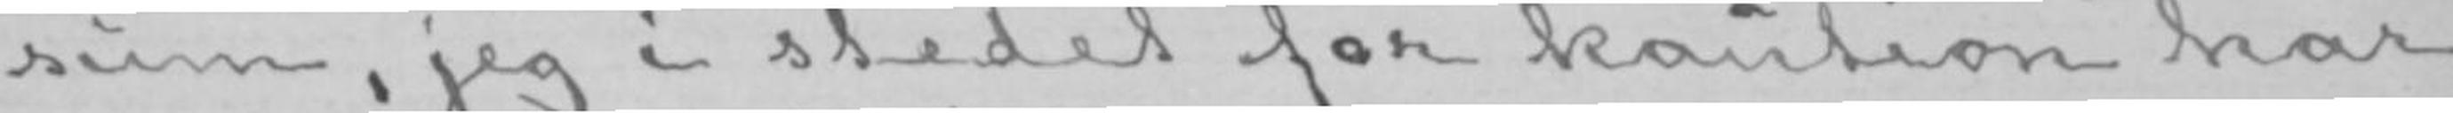sum, jeg i stedet for kaution har
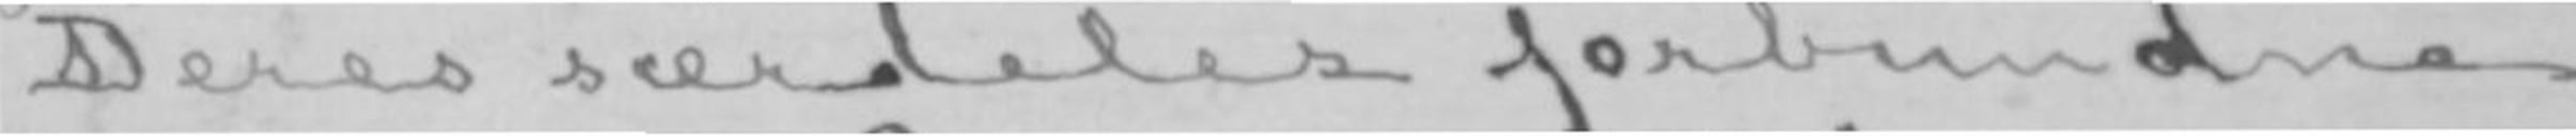Deres særdeles forbundne
